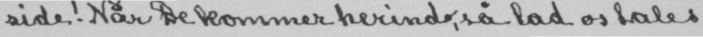side! Når De kommer herind., så lad os tales
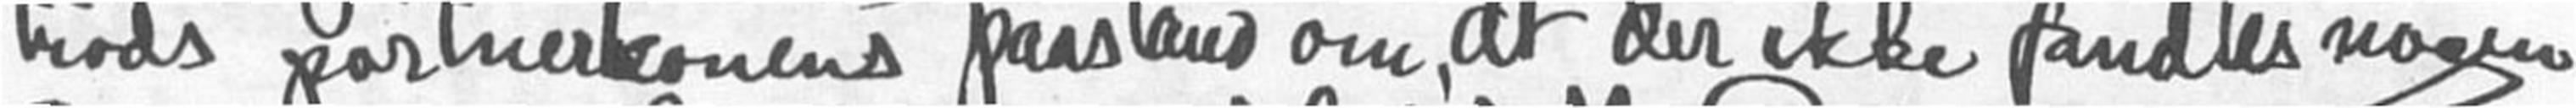trods portnerkonens paastand om at der ikke fandtes nogen
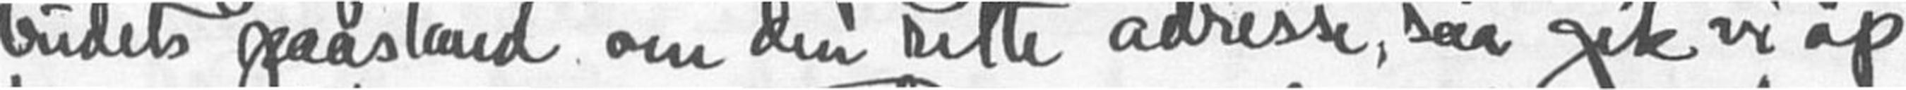budets paastand om den rette adresse, saa gik vi op
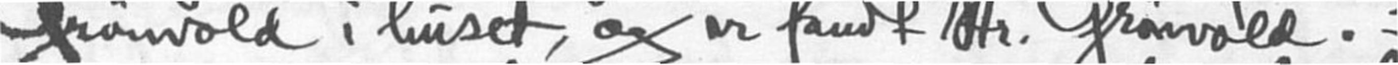Grønvold i huset, og vi fandt Hr. Grønvold.
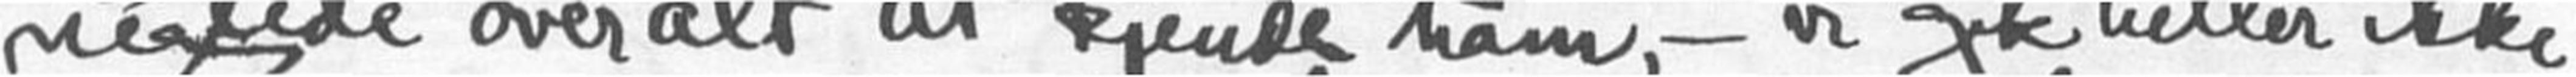negtede overalt at kjende ham, - vi gik heller ikke
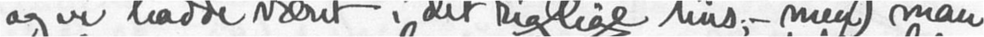og vi havde været i det rigtige hus, - men man
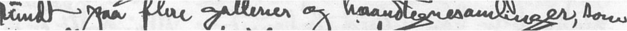rundt paa flere gallerier og haandtegnesamlinger, som
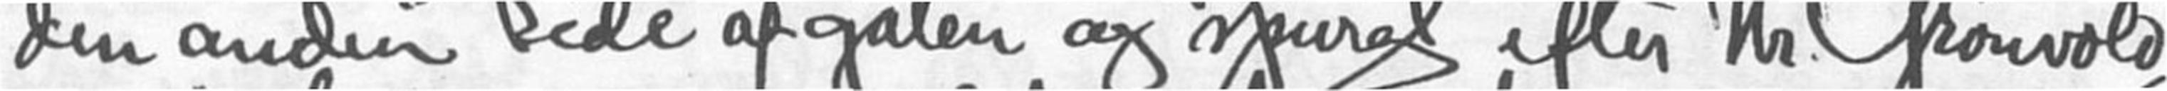den anden side af gaten og spurgt efter Hr. Grønvold,
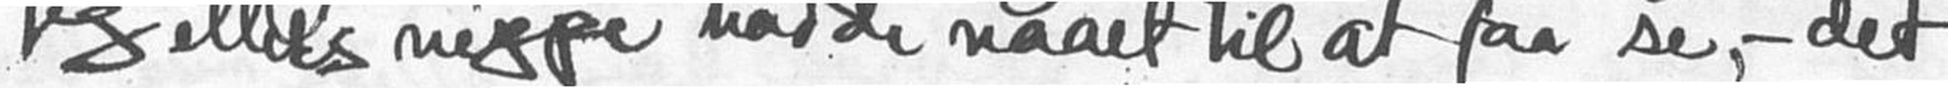jeg ellers neppe havde naaet til at faa se,- det
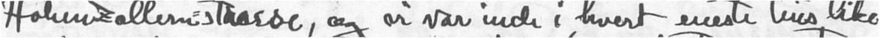Hohenzollernstrasse, og vi var inde i hvert eneste hus like
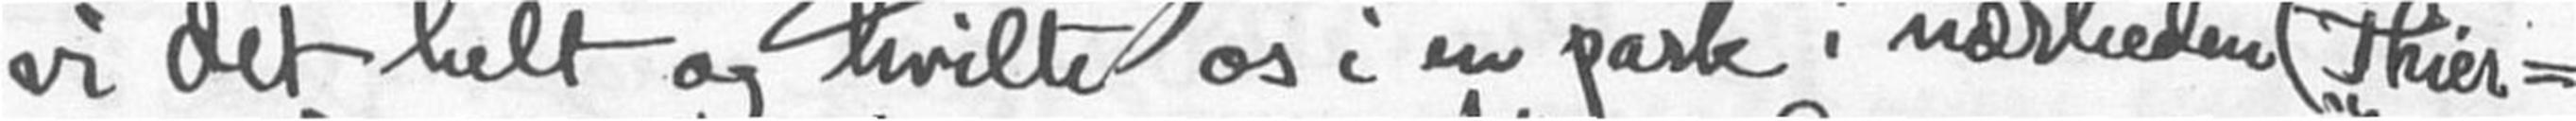vi det helt og hvilte os i en park i nærheden ("Thier-
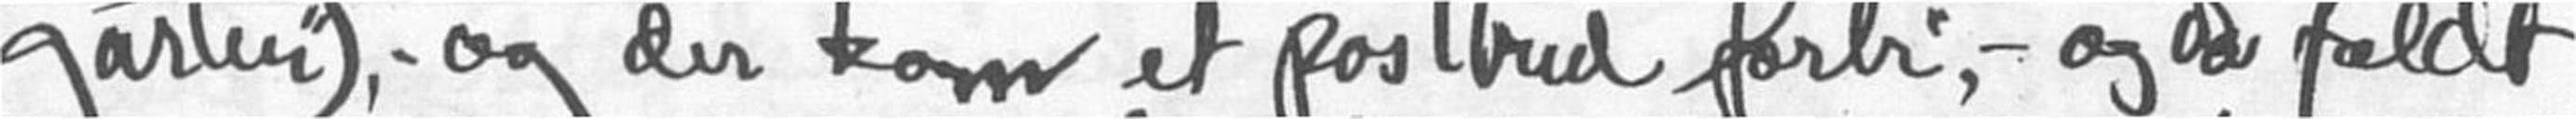garten") - og der kom et postbud forbi, - og da faldt
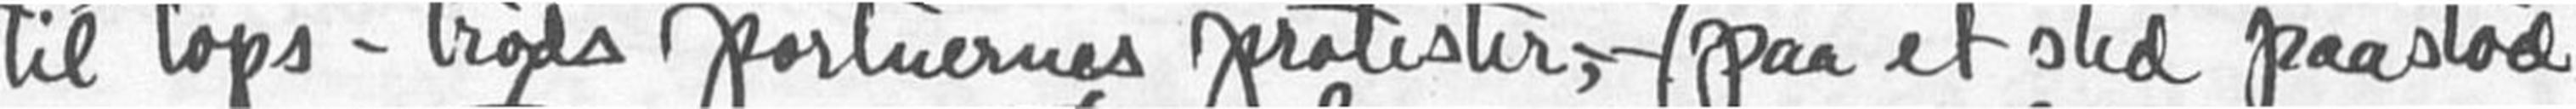til tops - trods portnernes protester, - (paa et sted paastod
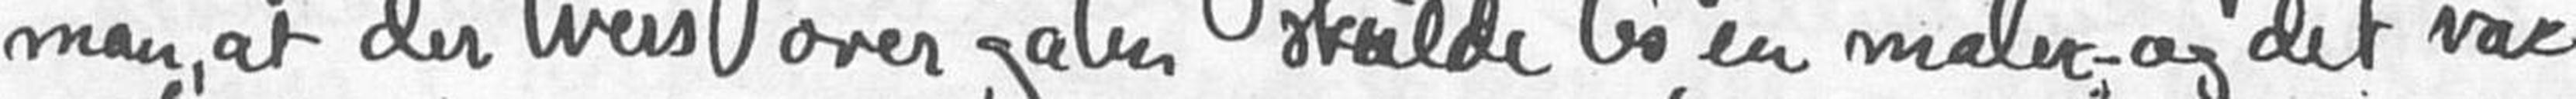man, at der tvers over gaten skulde bo en maler,- og det var
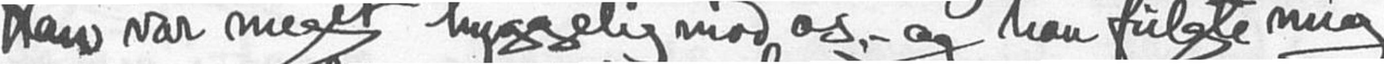Han var meget hyggelig mod os, - og han fulgte mig
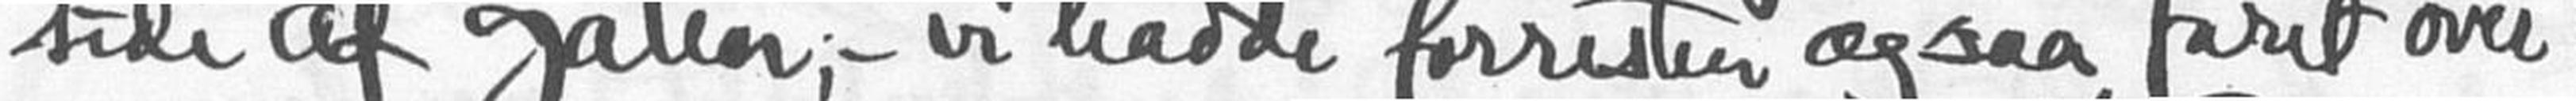side af gaten,- vi havde forresten ogsaa faret over
